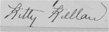Kitty Kielland.
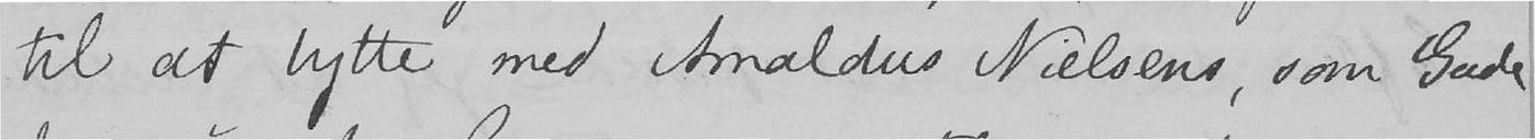til at bytte med Amaldus Nielsens, som Gude
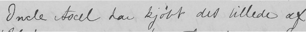Oncle Axel har kjøbt det billede af
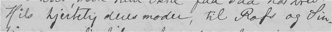Hils hjertelig deres moder, til Ross og Sin
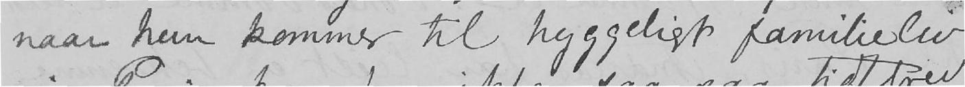naar hun kommer til hyggeligt familieliv
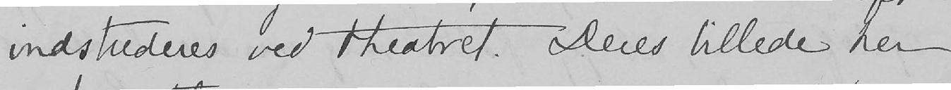indstuderes ved theatret. Deres billede her
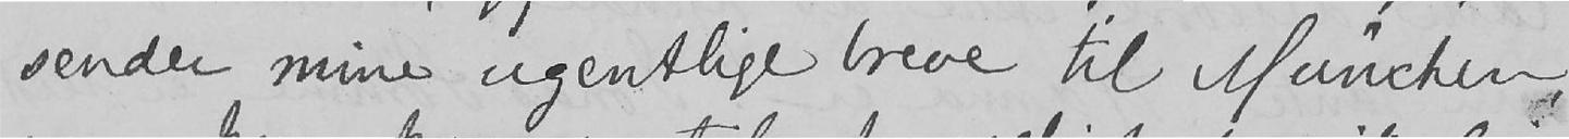sender mine ugentlige breve til München,
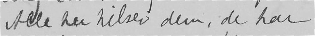Alle her hilser dem, de har
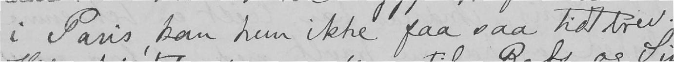i Paris, kan hun ikke faa saa tidt brev.
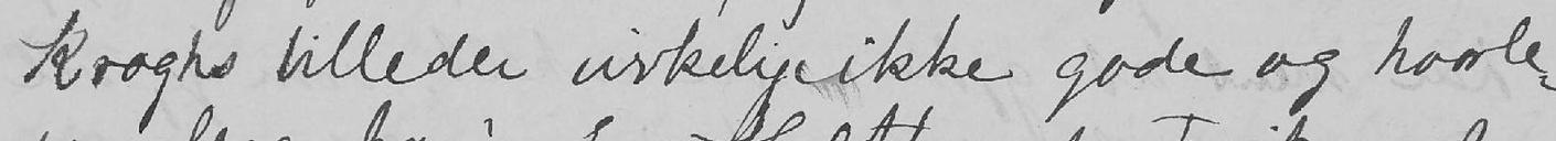Kroghs billeder virkelige ikke gode og hvorle-
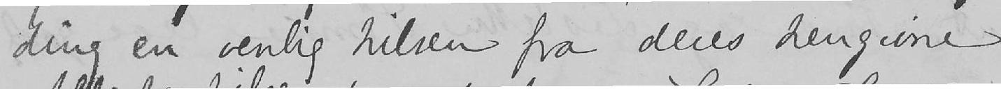ding en venlig hilsen fra deres hengivne
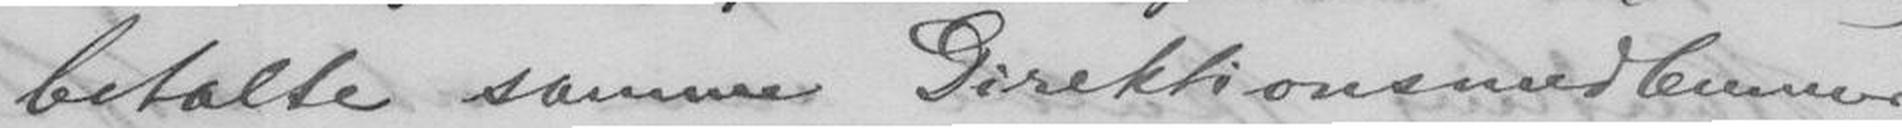betalte samme Direktionsmedlemmer
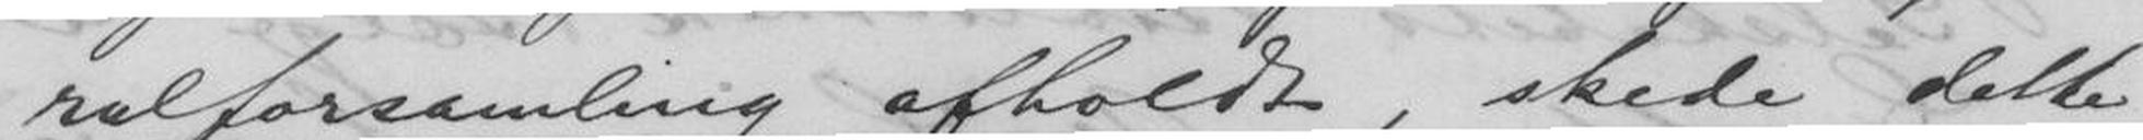ralforsamling afholdt, skede dette
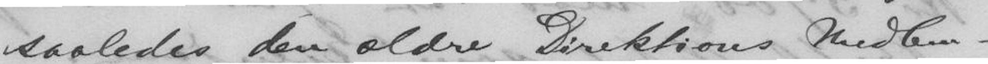saaledes den ældre Direktions Medlem-
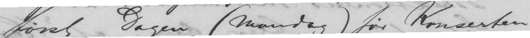først Dagen (Mandag) før Konserten
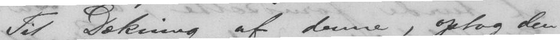Til Dækning af denne, optog den
